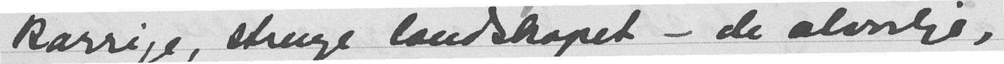Karrige, strenge landskapet - de alvorlige,
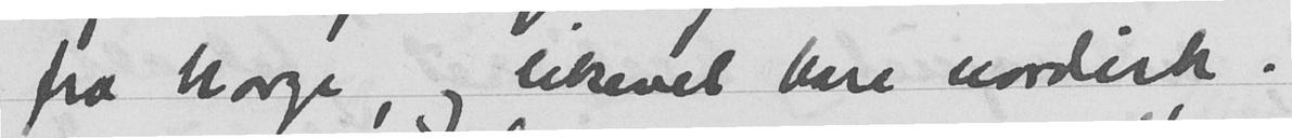fra Norge, og likevel bare nordisk.
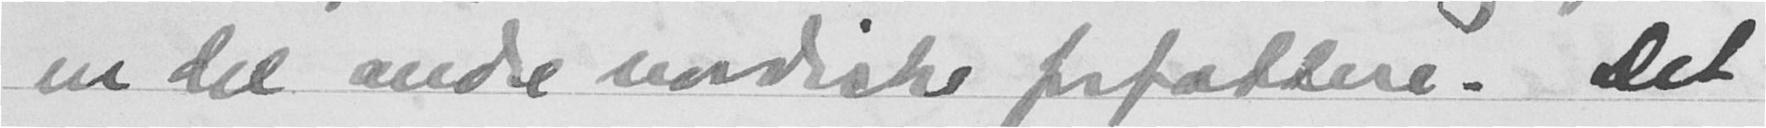en del andre nordiske forfattere. Det
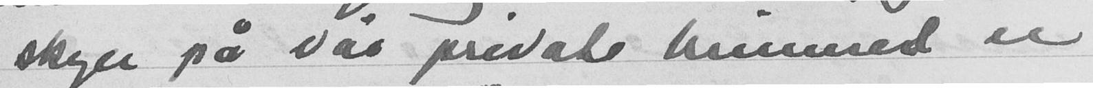skyer på var private himmel er
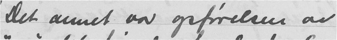Det annet var opførelsen av
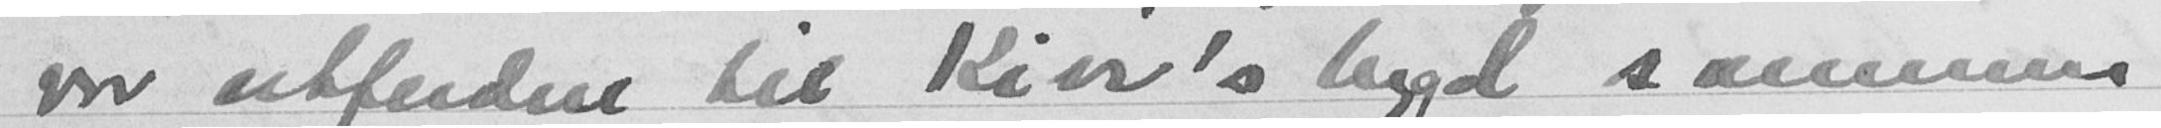var utferden til Kier's bygd sammen
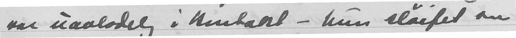var uavladelig i kontakt - hun sløifet med
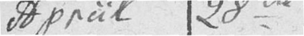Apriil 28de
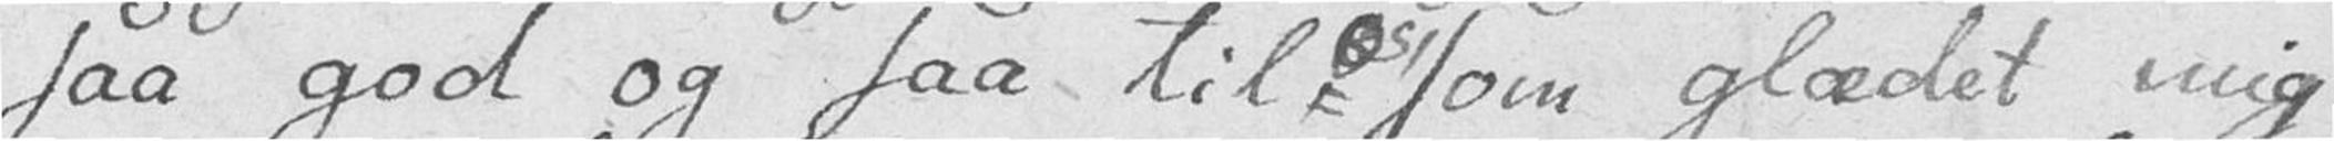saa god og saa til os, som glædet mig
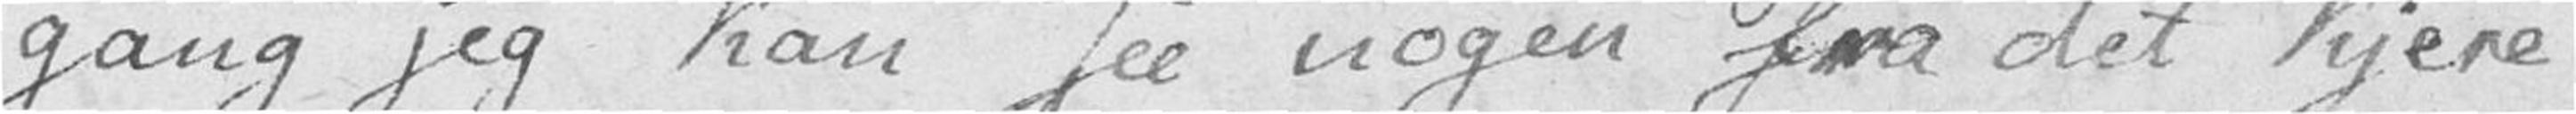gang jeg kan see nogen fra det kjere
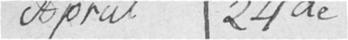Apriil 24de
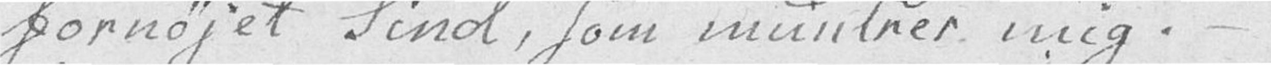fornøjet Sind, som muntrer mig. –
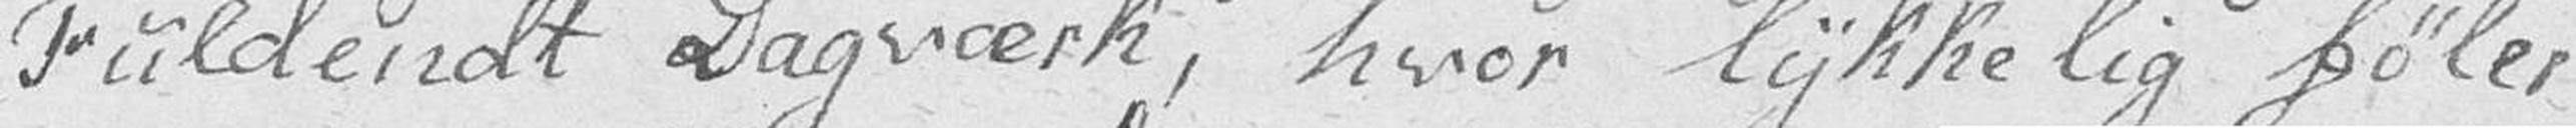Fuldendt Dagværk, hvor lykkelig føler
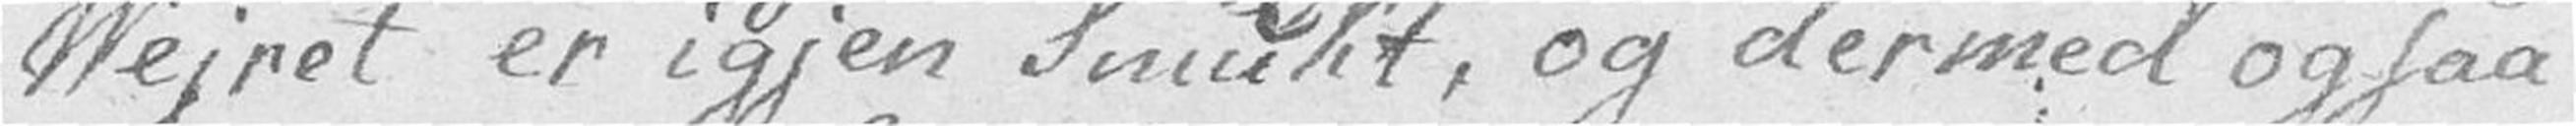Vejret er igjen Smukt, og dermed ogsaa
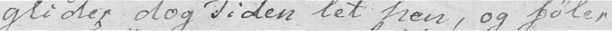glider dog Tiden let hen, og føler
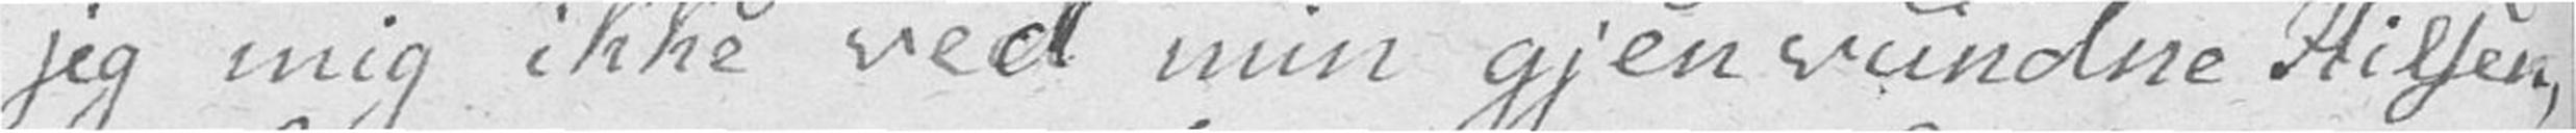jeg mig ikke ved min gjenvundne Hilsen,
